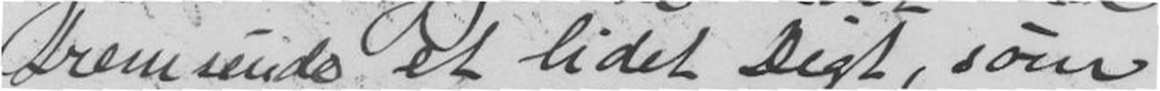frensende et lidet Digt, som
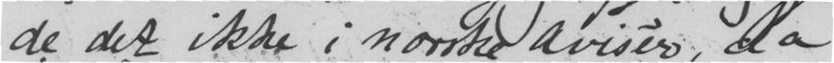de det ikke i norske Aviser, da
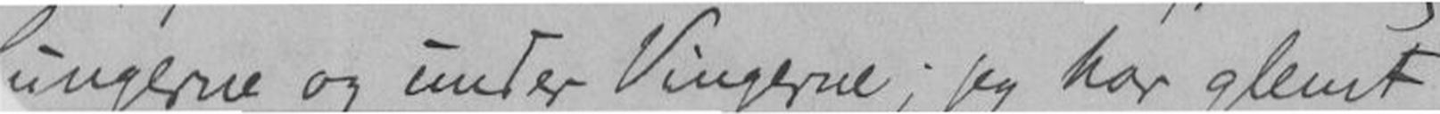Lungerne og under Vingerne; jeg har glemt
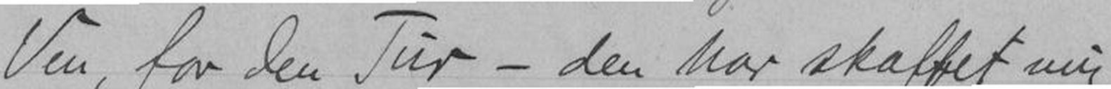Ven, for den Tur - den har skaffet mig
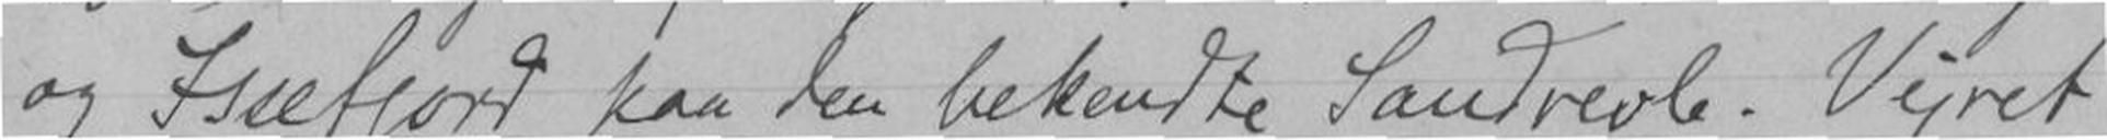og Itsefjord paa den bekendte Sandrevle. Vejret
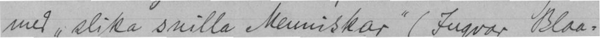med slika snilla Menniskar (Ingvar Blaa
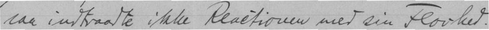saa indtraadte ikke Realtionen med sin Flovhed!
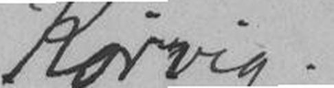Rørvig.
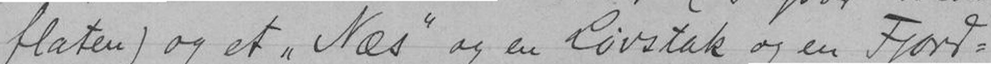flaten) og et Næs og en Løvstak og en Fjord=
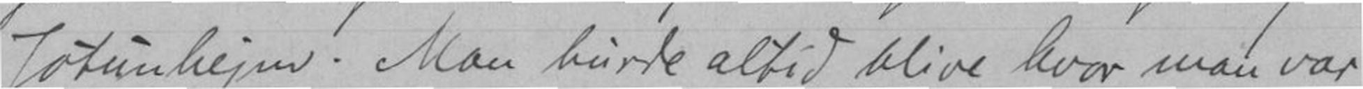Jotunhejm. Man burde altid blive hvor man var,
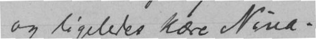og ligeledes kære Nina.
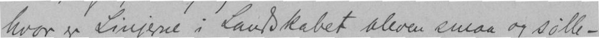hvor er Linjerne i Landskabet bleven smaa og sølle -
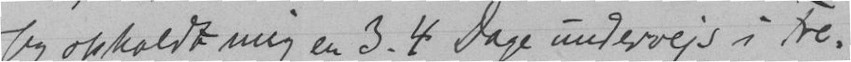Jeg opholdt mig en 3.4 Dage undervejs i Fre-
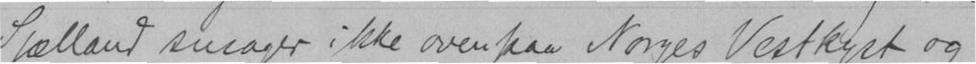Sjælland smager ikke ovenpaa Norges Vestkyst og
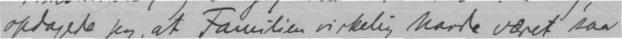opdagede jeg, at Familien virkelig havde været saa
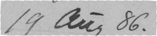19 Aug. 86
